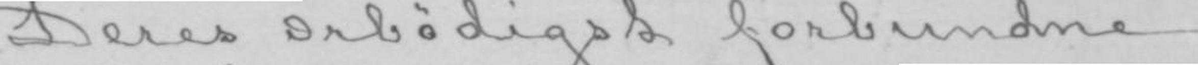Deres ærbødigst forbundne
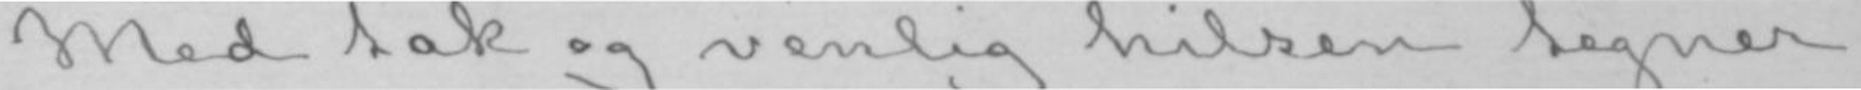Med tak og venlig hilsen tegner
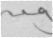jeg mig
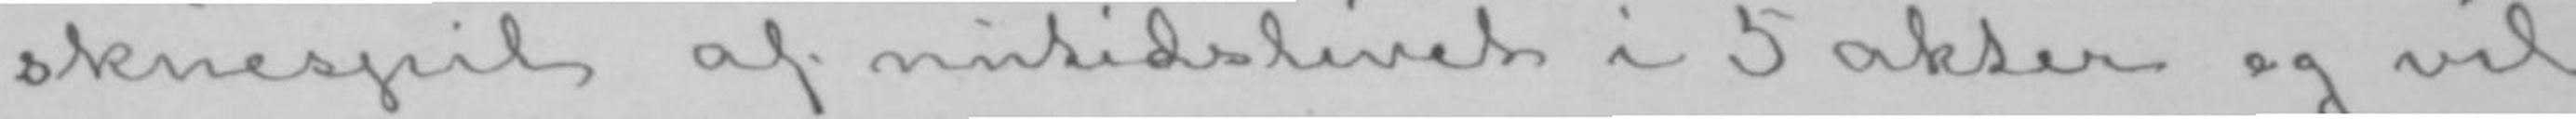skuespil af nutidslivet i 5 akter og vil
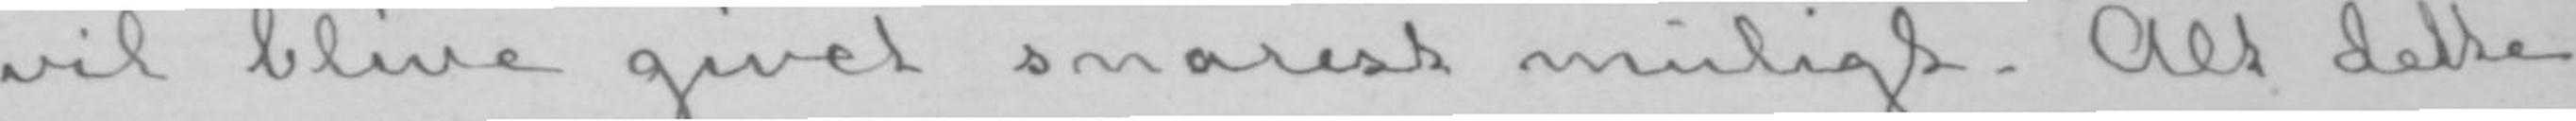vil blive givet snarest muligt. Alt dette
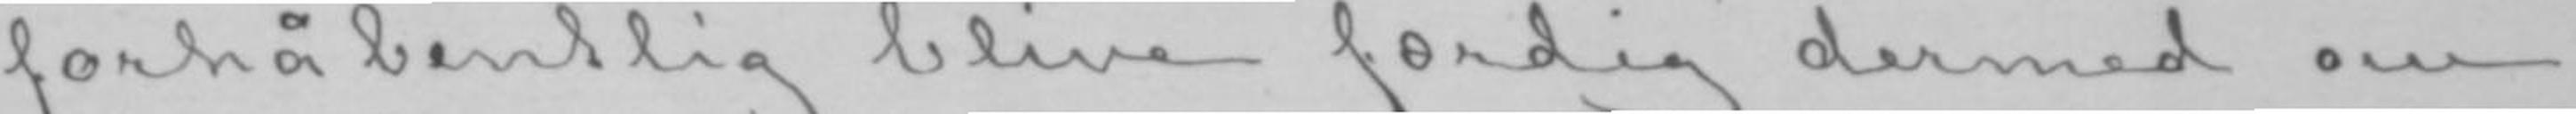forhåbentlig blive færdig dermed om
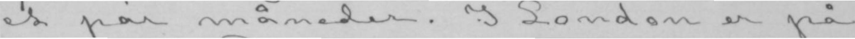et par måneder. I London er på
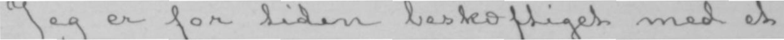Jeg er for tiden beskæftiget med et
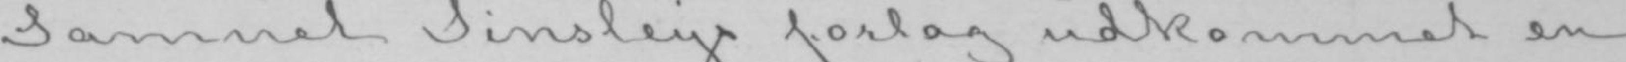Samuel Tinsleys forlag udkommet en
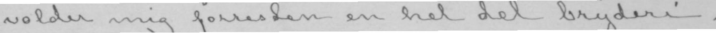volder mig forresten en hel del bryderi.
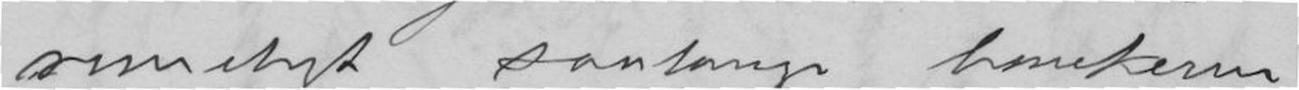rimeligt saalange bankerne
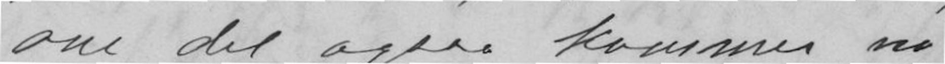om det ogsaa kommer no-
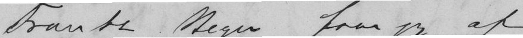Frantz Beyer faar jeg af
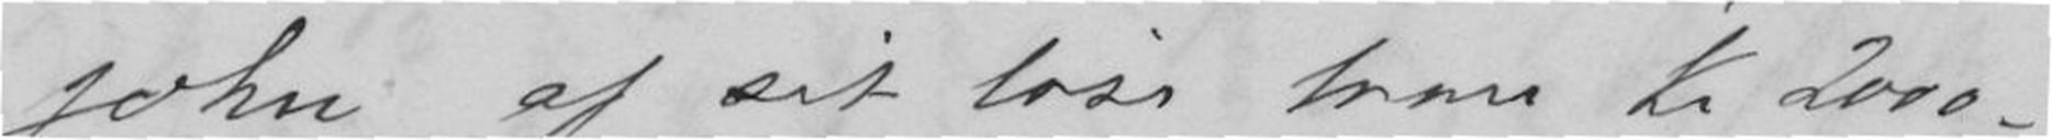John af sit løse krav kr 2000.-
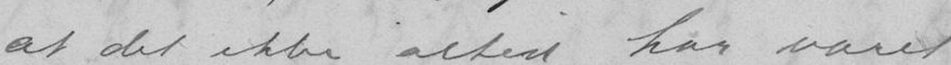at det ikke altid har været,
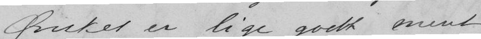Ønsket er lige godt ment,
